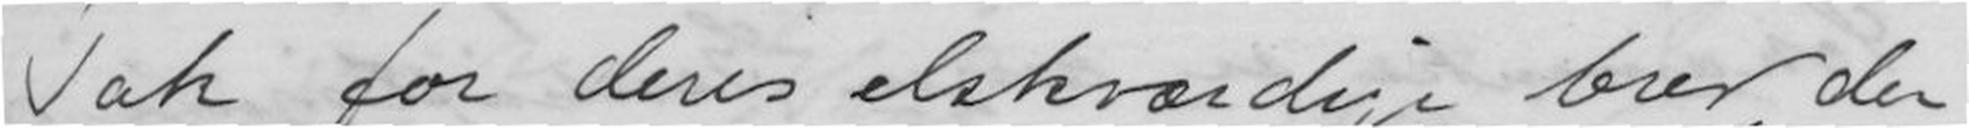Tak for deres elskværdige brev, der
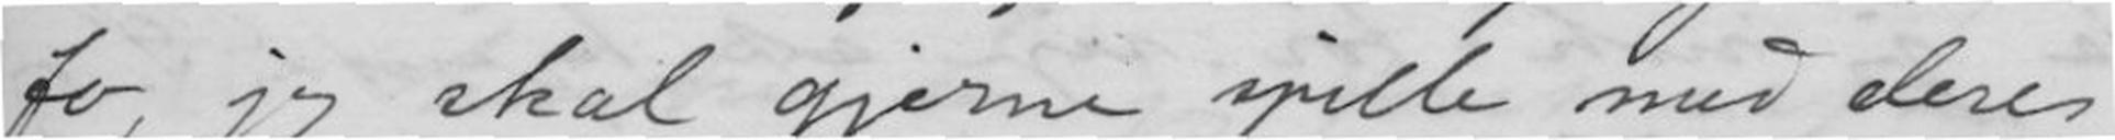Jo, jeg skal gjerne spille med deres
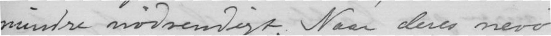mindre nødvendigt. Naar deres nevø
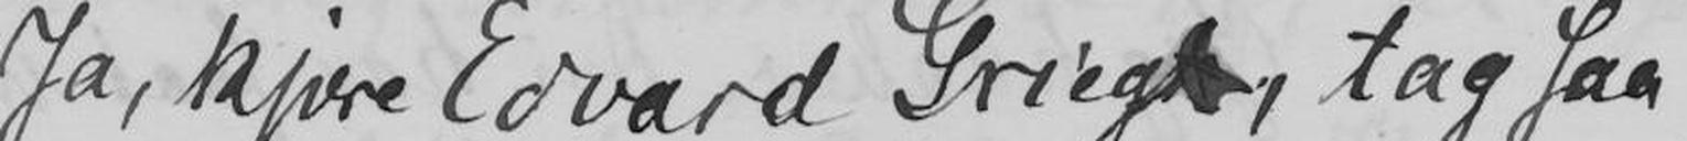Ja, kjære Edvard Grieg, tag saa
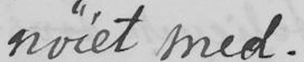nøiet med.
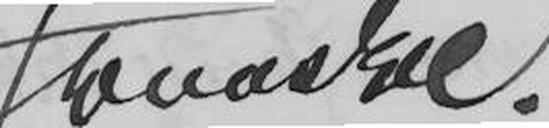Jonas Lie.
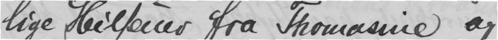lige Hilsener fra Thomasine og
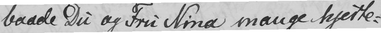baade Du og Fru Nina mange hjerte-
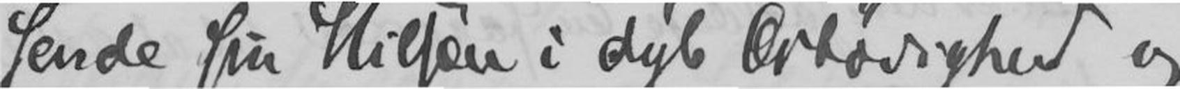sende sin Hilsen i dyb Ærbødighed og
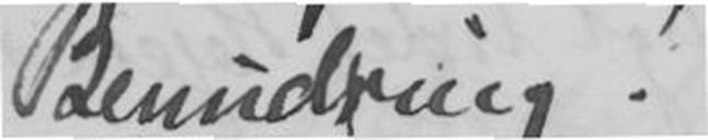Beundring!
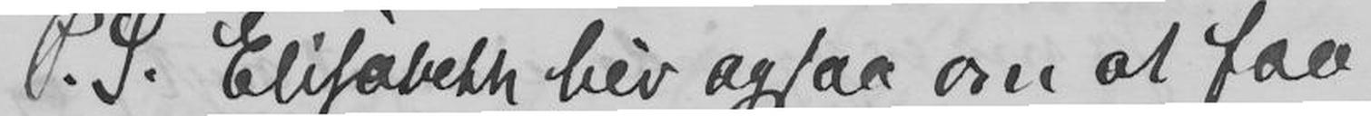P. S. Elisabeth ber ogsaa om at faa
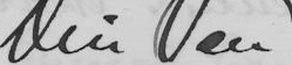Din Ven
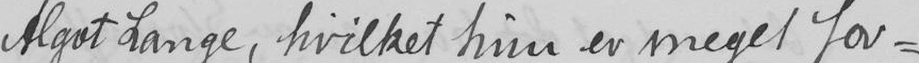Algot Lange, hvilket hun er meget for-
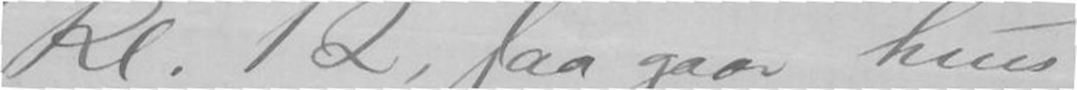Kl. 12, saa gaar hun
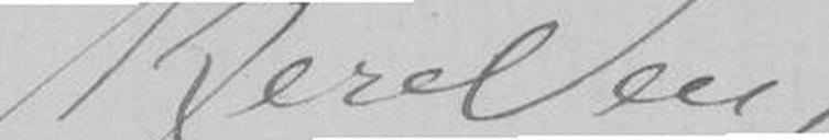Kjere Ven,
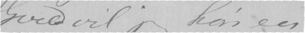Ikveld vil jeg høre en
